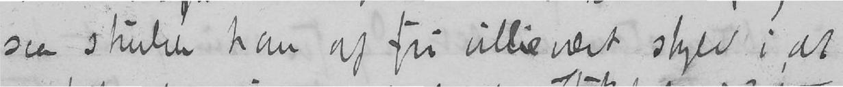saa skulde han af fri villie vært skyld i, at
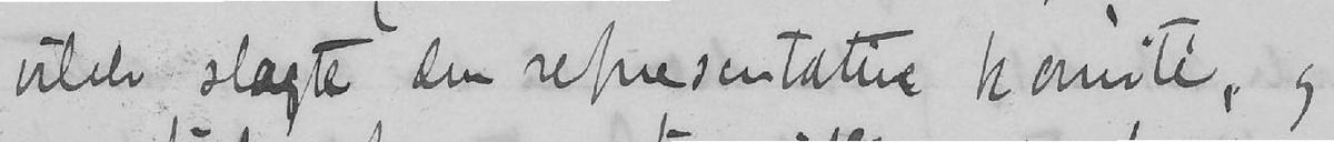vilde slagte den representative komité, og
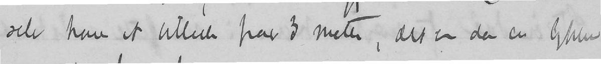selv have et billede paa 3 meter, det er da en lykke
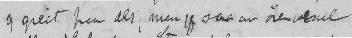og greit paa det, men jeg saa en øienvexel
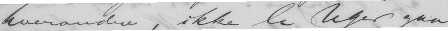hverandre, ikke la Uger gaa
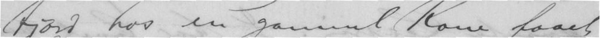fjord hos en gammel Kone faaet
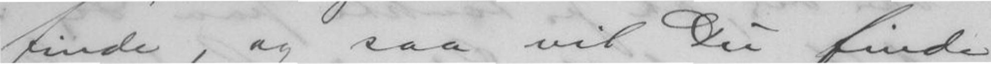finde, og saa vil Du finde
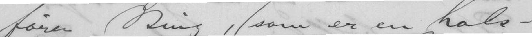fører Bing, (som er en hals-
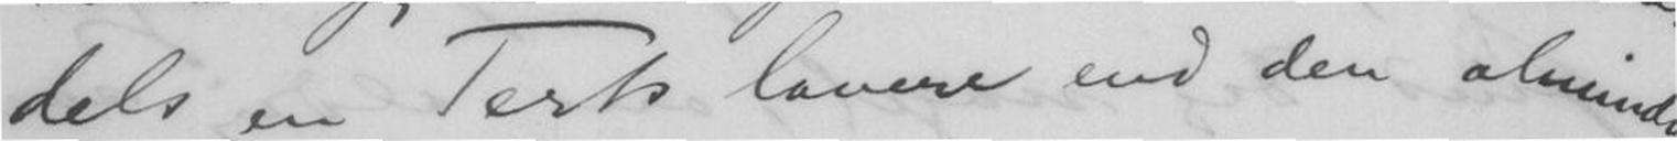dels en Terts lavere end den almindelige
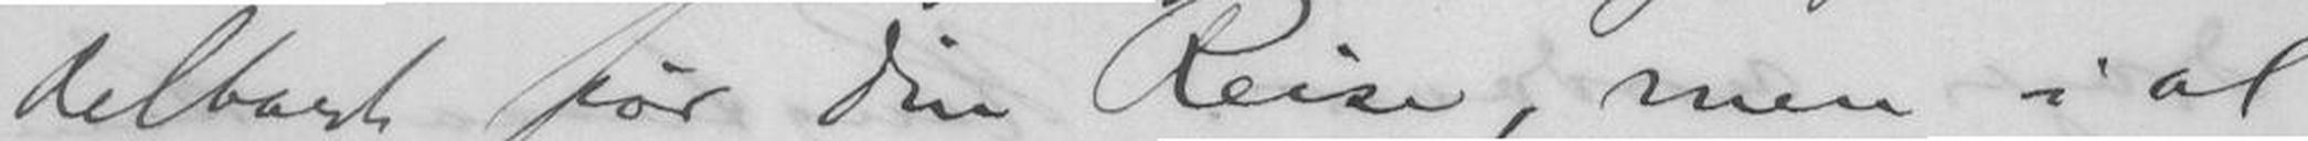delbart før din Reise, men i al
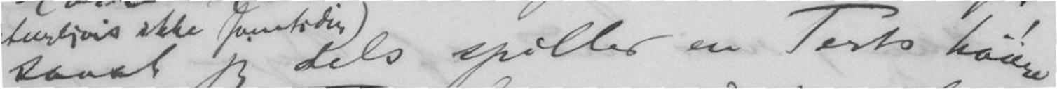saaat jeg dels spiller en Terts høiere,
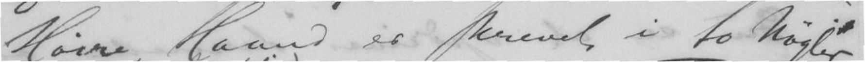Høire Haand er skrevet i to Nøgler,
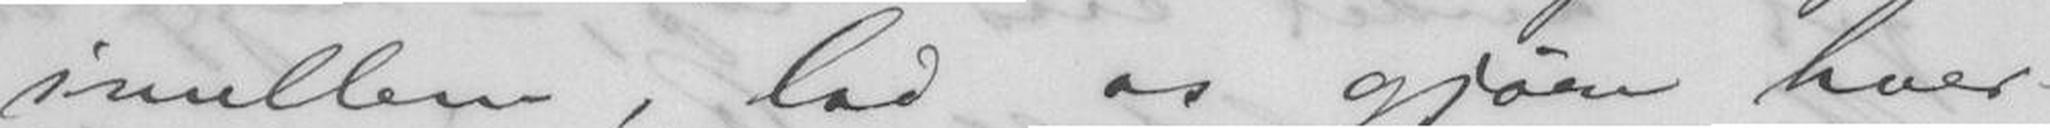imellem, lad os gjøre hver-
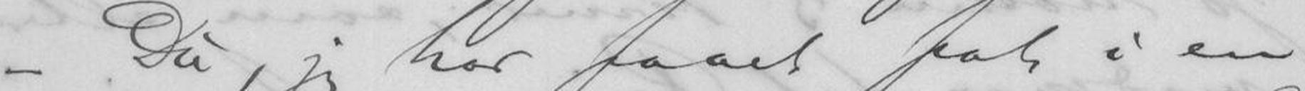- Du, jeg har faaet fat i en
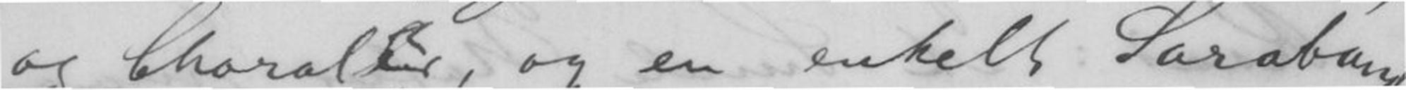og Choraler, og en enkelt Sarabande.
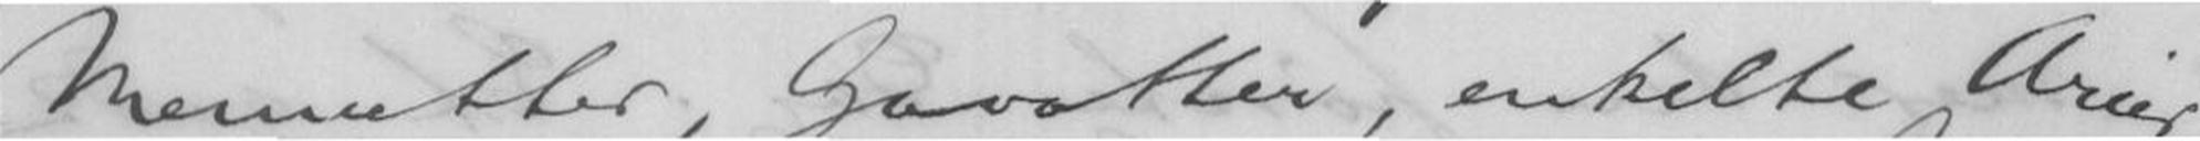Menuetter, Gavotter, enkelte Arier,
